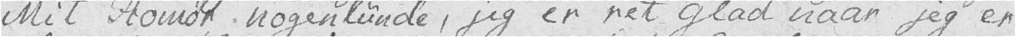Mit Homør nogenlunde, jeg er ret glad naar jeg er
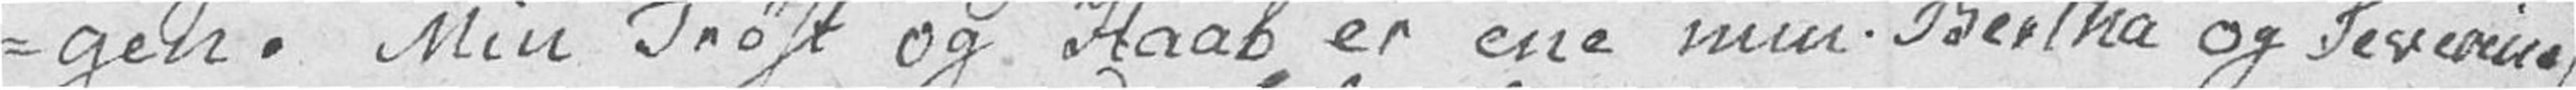-gen. Min Trøst og Haab er ene min Bertha og Severine,
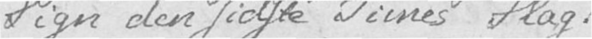Sign den sidste Times Slag!
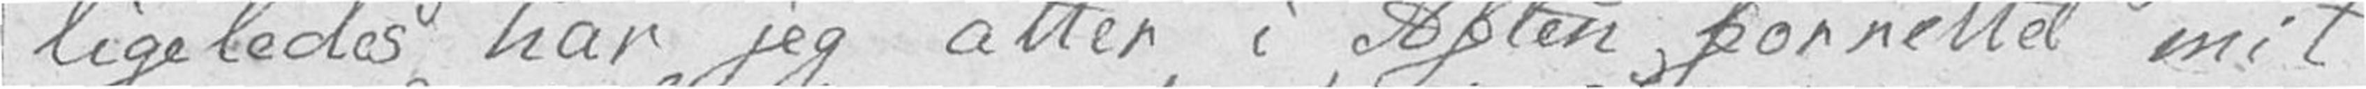ligeledes har jeg atter i Aften forrettet mit
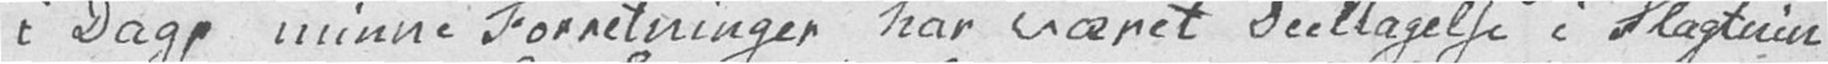i Dag, minne Forretninger har været Deeltagelse i Slagtnin
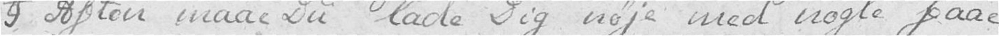I Aften maae Du lade Dig nøje med nogle faae
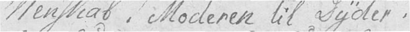Venskab! Moderen til Dyder!
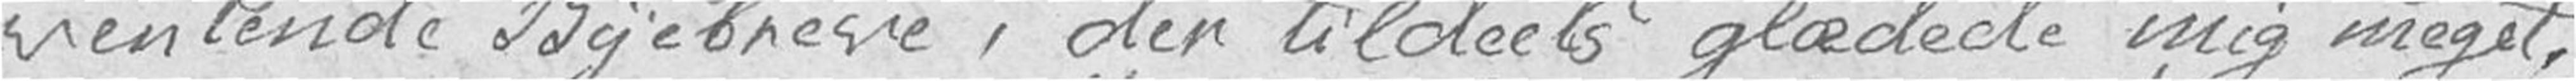ventende Byebreve, der tildeels glædede mig meget,
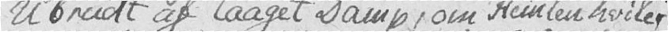ubrudt af taaget Damp, om Himlen hviler!
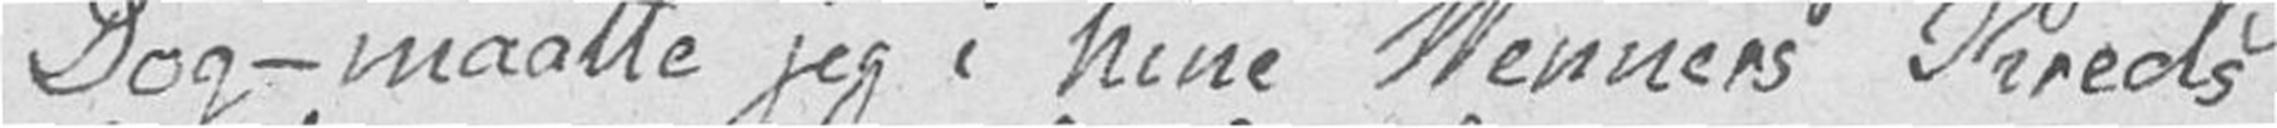Dog – maatte jeg i hine Venners Kreds
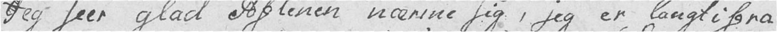Jeg seer glad Aftenen nærme sig, jeg er langtifra
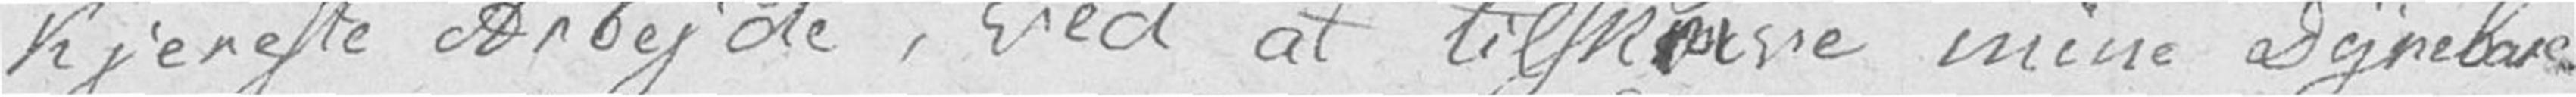kjereste Arbejde, ved at tilskrive mine Dyrebare
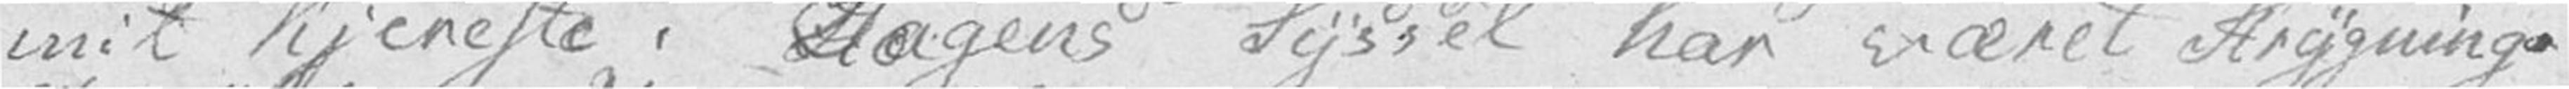mit kjereste. Dagens Syssel har været Strygning.
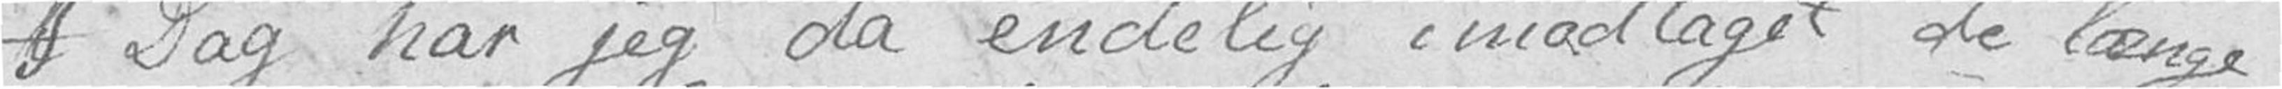I Dag har jeg da endelig imodtaget de længe
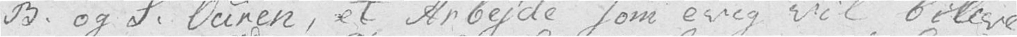B. og S. Ouren, et Arbejde som evig vil blive
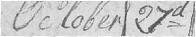October 27d
